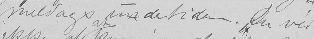meldags undertiden. "Du véd
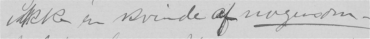ikke en kvinde af nogensom-
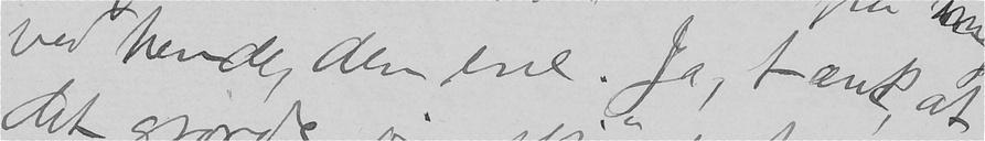ved hende, den ene. Ja, tænk at
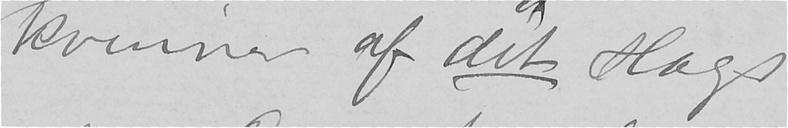kvinner af det slags
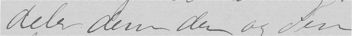deler dem den og den
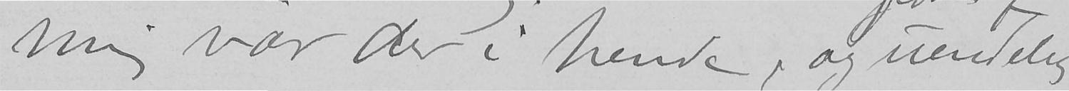mig var der i hende, og uendelig
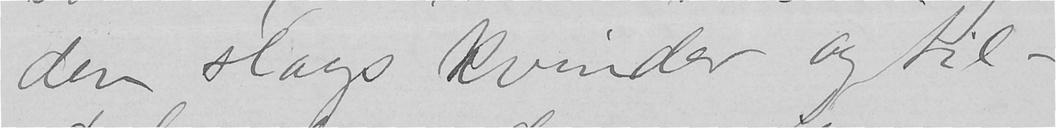den slags kvinder og til-
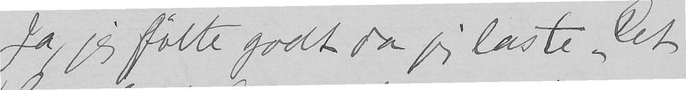Ja, jeg følte godt da jeg læste "Det
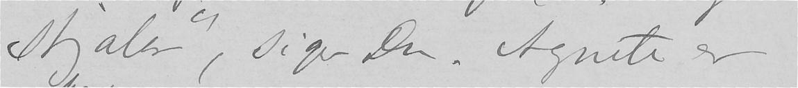stjæler", siger Du. Agnete er
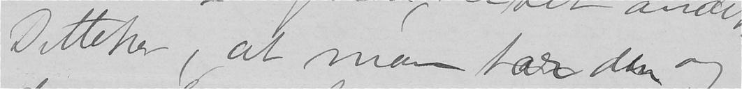Detteher, at man tar den og
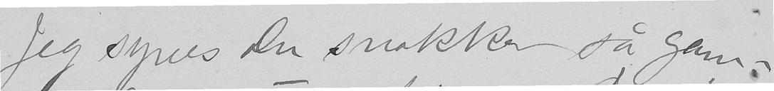Jeg synes Du snakker så gam-
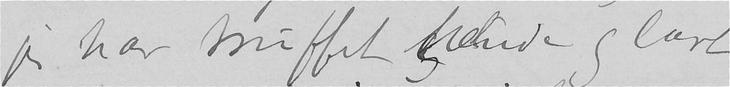jeg har truffet hende og lært
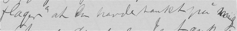flager" at Du havde tænkt på mig
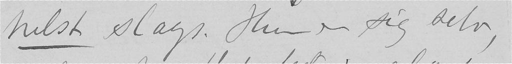helst slags. Hun er sig selv,
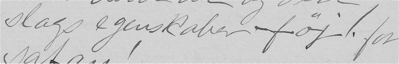slags egenskaber - føj! for
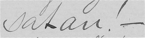satan! -
